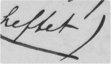heftet)
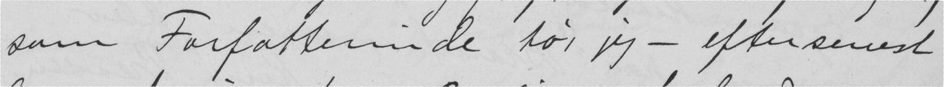som Forfatterinde tør jeg - eftersenest
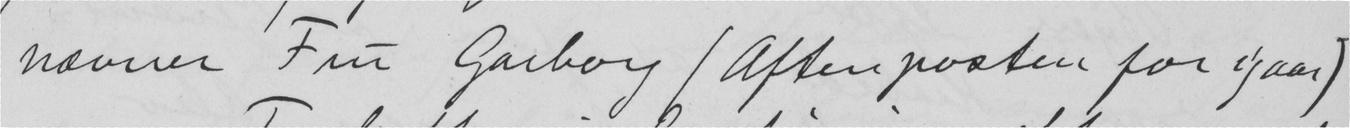nævner Fru Garborg (Aftenposten for igaar)
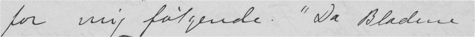for mig følgende. "Da Bladene
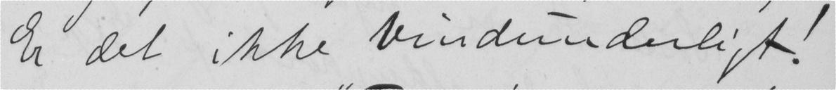Er det ikke vindunderligt!
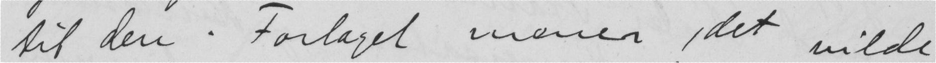til den. Forlaget mener, det vilde
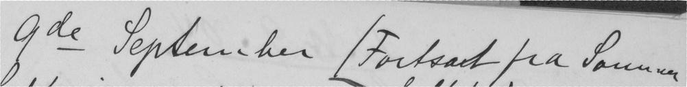9de September (Fortsat fra Sommer
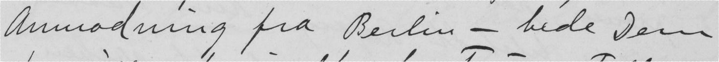Anmodning fra Berlin — bede Dem
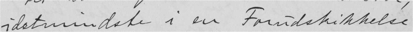idetmindste i en Forudskikkelse
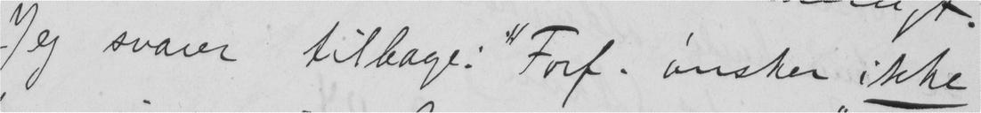Jeg svarer tilbage: "Forf. ønsker ikke
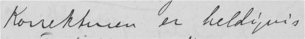Korekturen er heldigvis
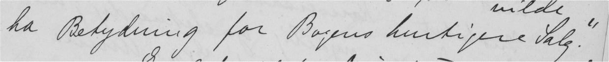ha Betydning for Bogens hurtigere Salg."
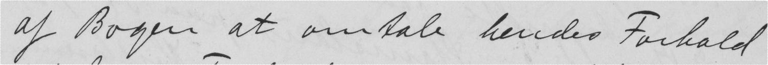af Bogen at omtale hendes Forhold
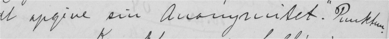at opgive sin Anonymitet." Punktum.
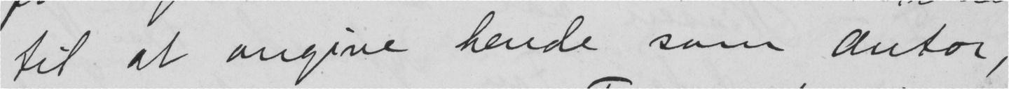til at angive hende som autor,
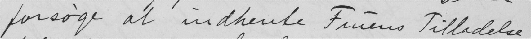forsøge at indhente Fruens Tilladelse
